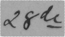28de
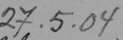27.5.04
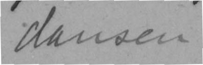dansen
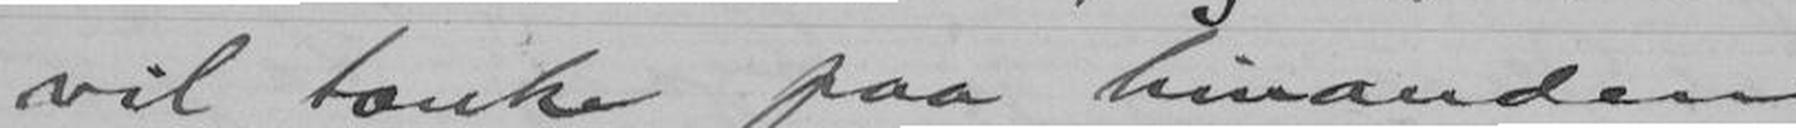vil tænke paa hinanden
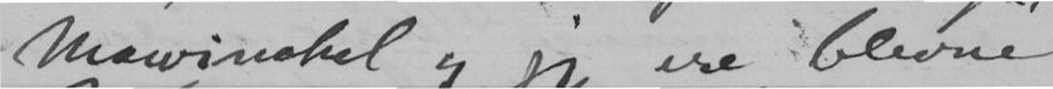Mowinckel og jeg ere blevne
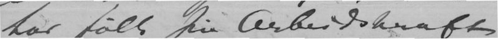har følt sin Arbeidskraft
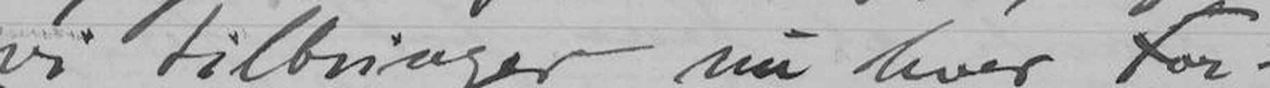vi tilbringer nu hver For-
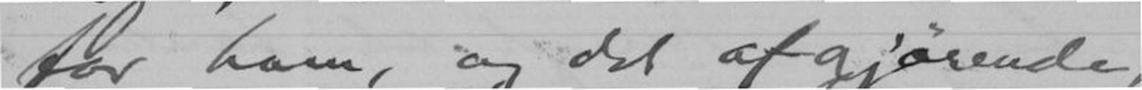for ham, og det afgjørende,
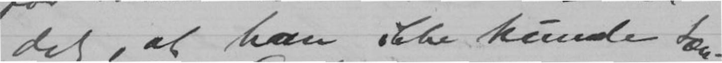det, at han ikke kunde tæn-
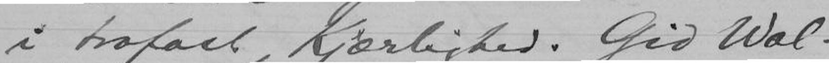i trofast kjærlighed. Gid Wal-
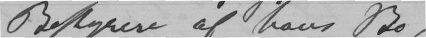Bestyrere af hans Bo,
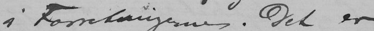i Forretningerne. Det er
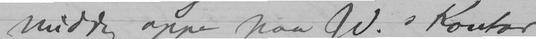middag oppe paa W.s Kontor
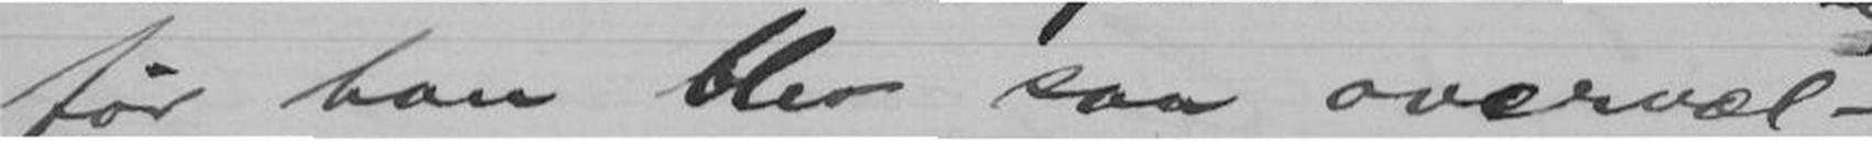før han blev saa overvæl-
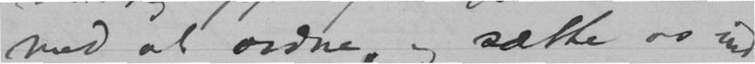med at ordne, og sætte os ind
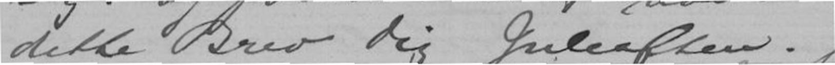dette Brev Dig Juleaften. Jeg
